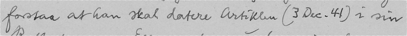forstaa at han skal datere Artiklen (3 Dec. 41) i sin
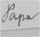Papa
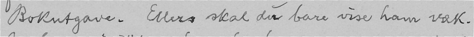Bokutgave. Ellers skal du bare vise ham væk.
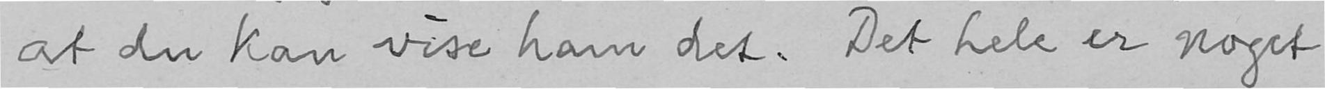at du kan vise ham det. Det hele er noget
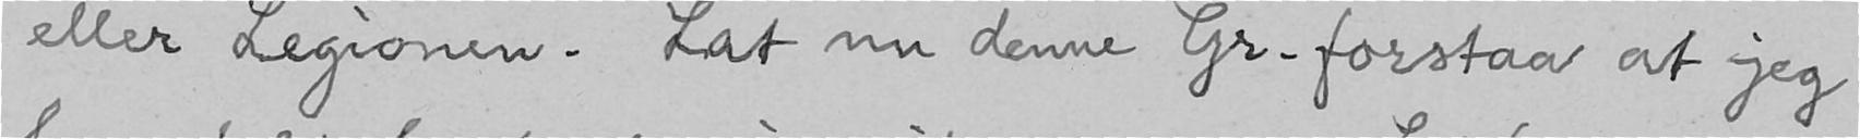eller Legionen. Lat nu denne Gr. forstaa at jeg
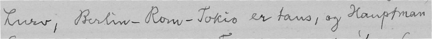Lurv, Berlin-Rom-Tokio er taus, og Hauptman
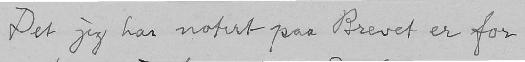Det jeg har notert paa Brevet er for
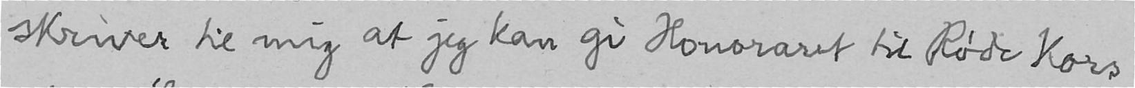skriver til mig at jeg kan gi Honoraret til Røde Kors
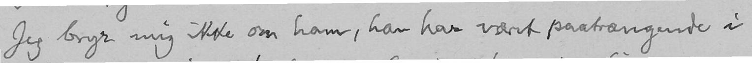Jeg bryr mig ikke om ham, han har været paatrængende i
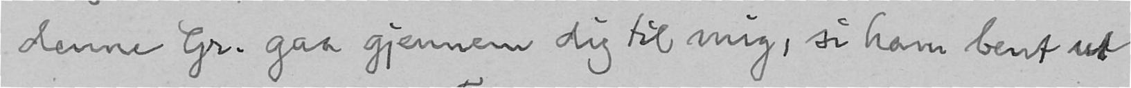denne Gr. gaa gjennem dig til mig, si ham bent ut
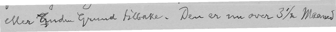eller anden Grund tilbake. Den er nu over 3 1/2 Maaned
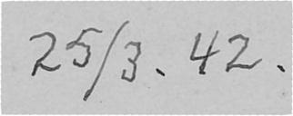25/3. 42.
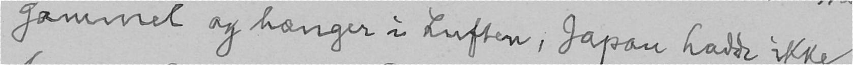gammel og hænger i Luften, Japan hadde ikke
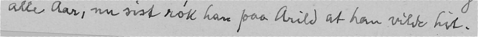alle Aar, nu sist røk han paa Arild at han vilde hit.
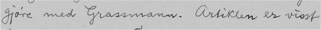gjøre med Grassmann. Artiklen er visst
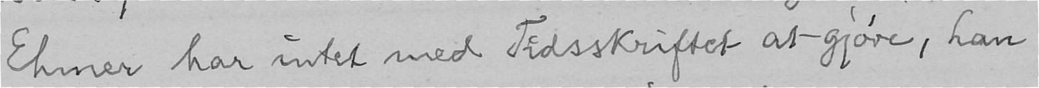Ehmer har intet med Tidsskriftet at gjøre, han
